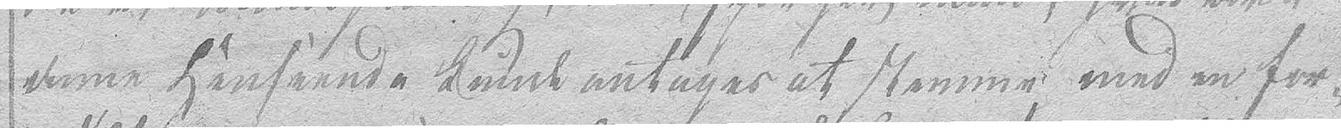denne Henseende kunde antages at stemme med en for-
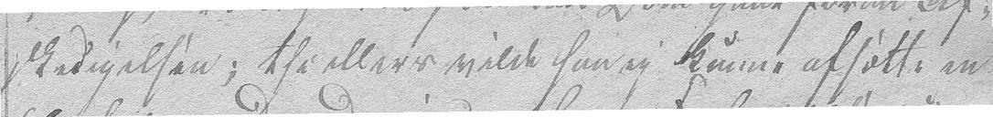skedigelsen; thi ellers vilde han ey kunne afsætte en
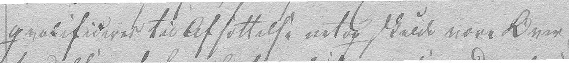qvalifisere til Afsættelse netop skulde være Over-
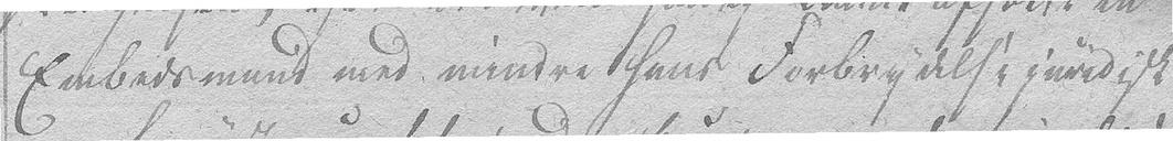Embedsmand med mindre hans Forbrydelse juridisk
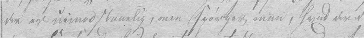der er uimodstaaelig, men spørger man, hvad der i
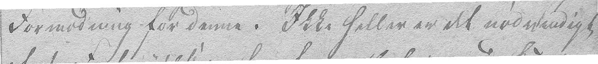Formodning for denne. Ikke heller er det nødvendigt,
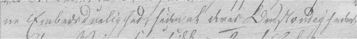ne Embedsduelighed, siden at deres Omstændigheder
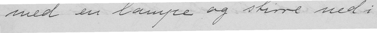med en lampe og stirre ned i
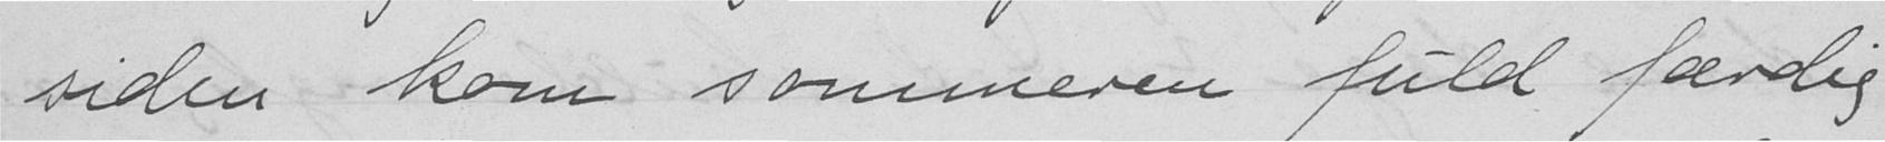siden kom sommeren fuld færdig
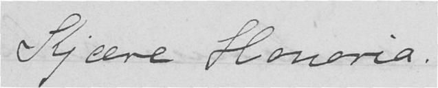Kjære Honoria!
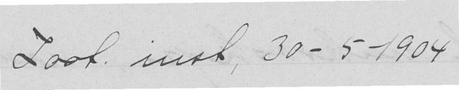Zoot. inst, 30-5-1904
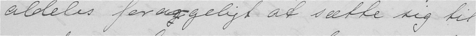aldeles forargeligt at sætte sig til
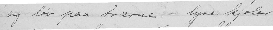og løv paa trærne, - lyse kjoler
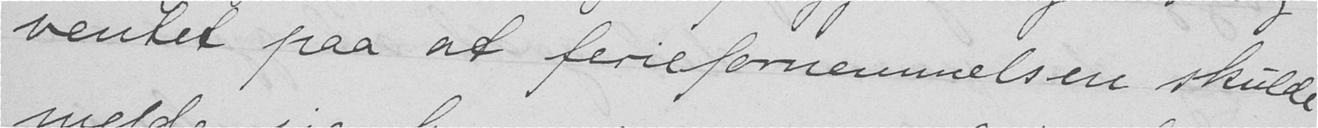ventet paa at feriefornemmelsen skulde
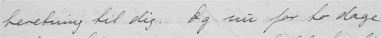beretning til dig. Og nu for to dage
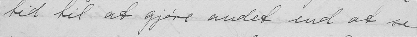tid til at gjøre andet end at se
